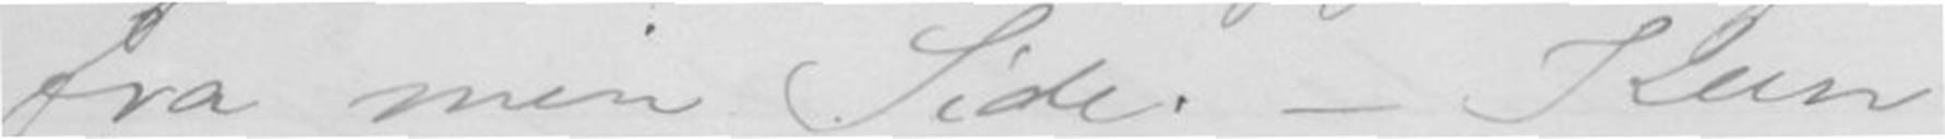fra min Side. - Kun
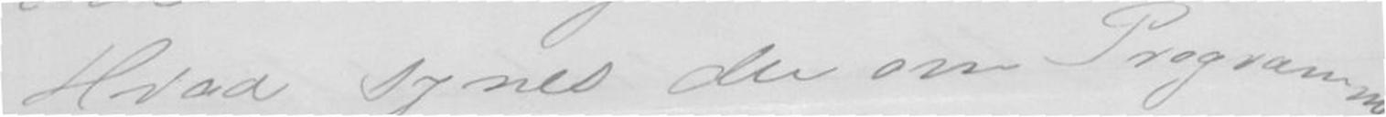Hvad synes du om Programmet?
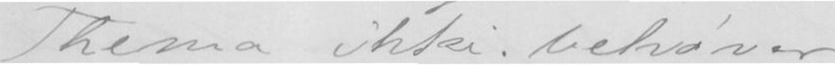Thema ikke behøver
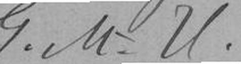G. M=H.
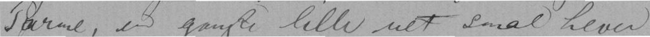Tarme, en ganske lille net smal Lever
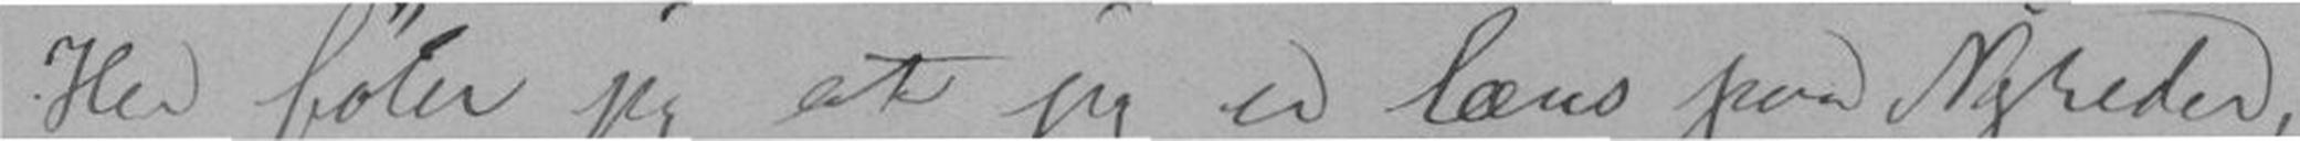Her føler jeg at jeg er læns paa Nyheder,
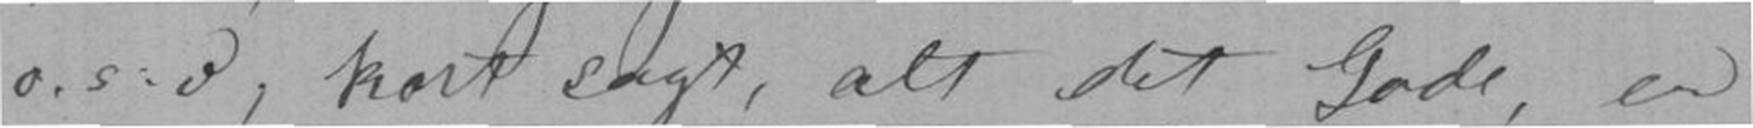o.s.v., kort sagt, alt det Gode, en
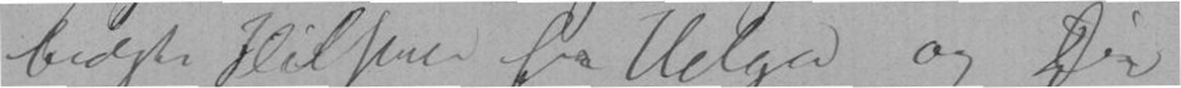bedste Hilsener fra Helga og Din
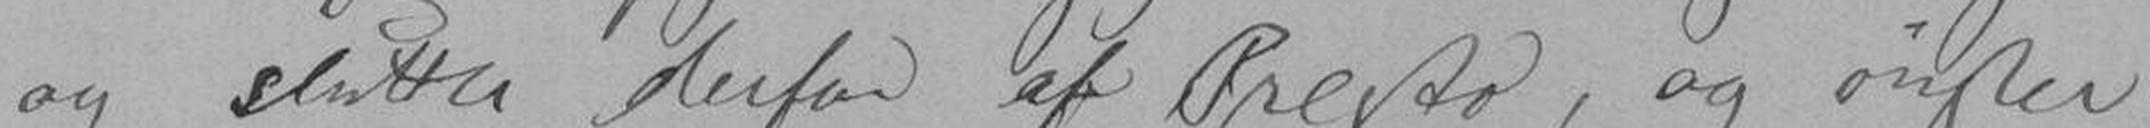og slutter derfor af Presto, og ønsker
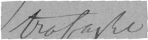trofaste
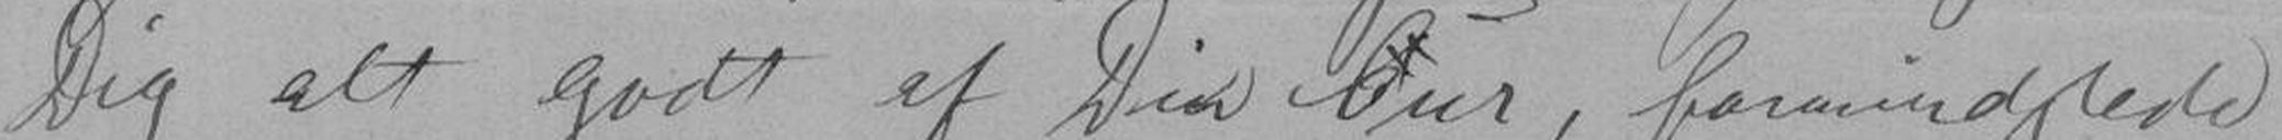Dig alt godt af Din Cur, formindskede
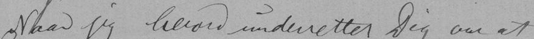Naar jeg herved underretter Dig om at
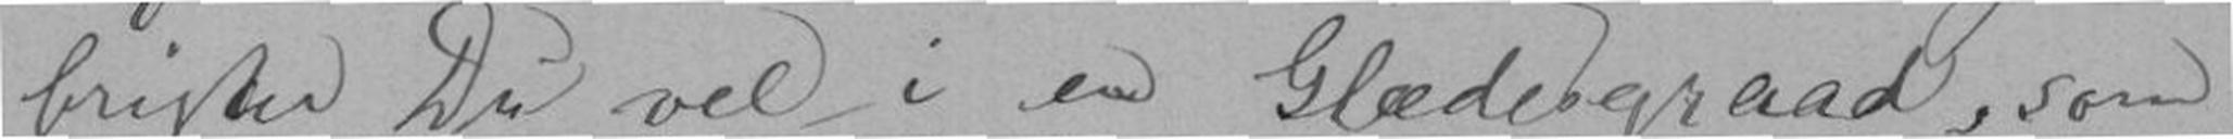brister Du vel - i en Glædesgraad, som
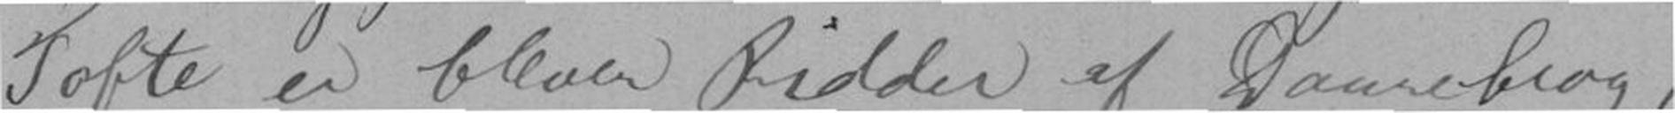Tofte er bleven Ridde af Danneborg,
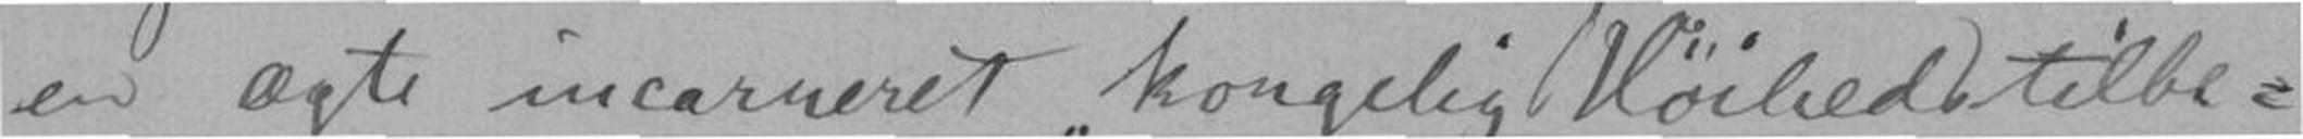en ægte incarneret kongelig Høihedstilbe-
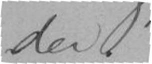der.
